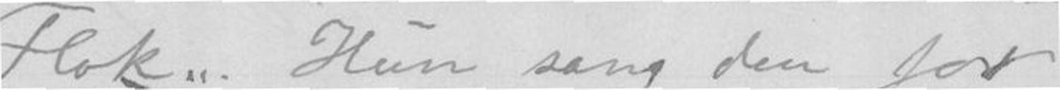Flok" Hun sang den for
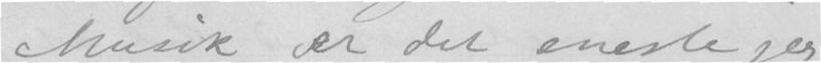Musik er det eneste jeg
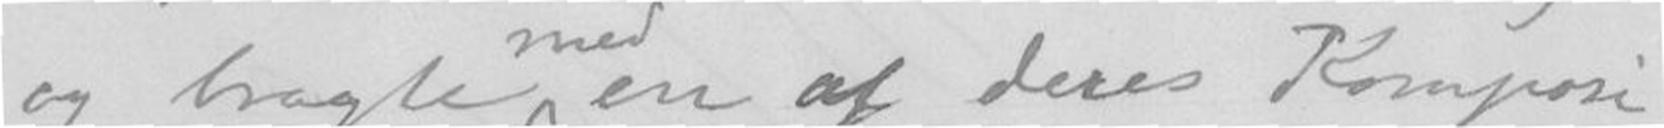og bragte med en af deres Komposi
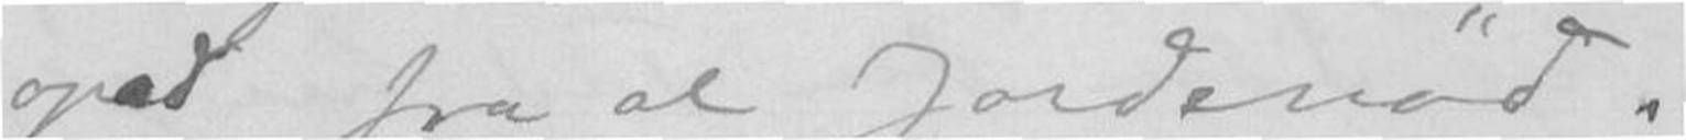opad fra al Jordenød.
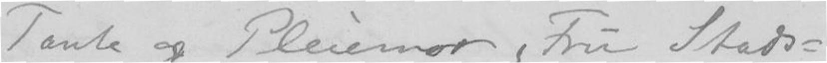Tante og Pleiemor, Fru Stads-
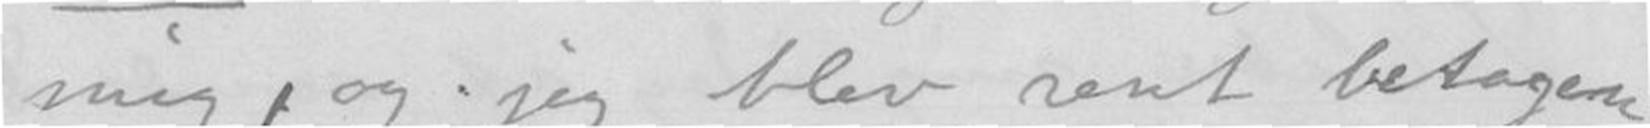mig, og jeg blev rent betagen
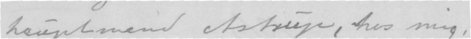hauptmand Astrup, hos mig
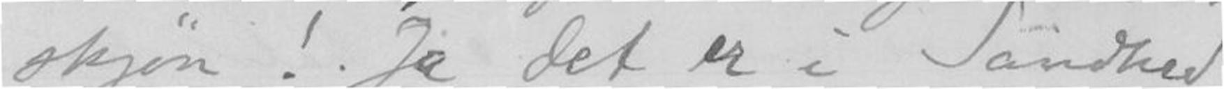skjøn! Ja det er i Sandhed
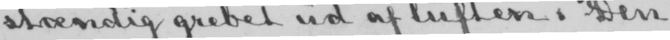stændig grebet ud af luften. Den
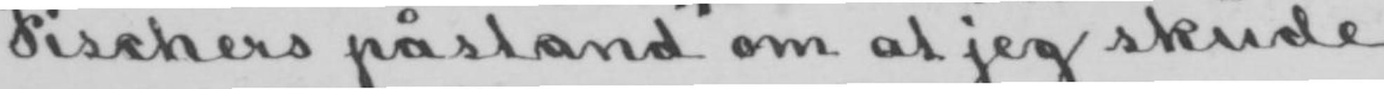Fischers påstand om at jeg skude
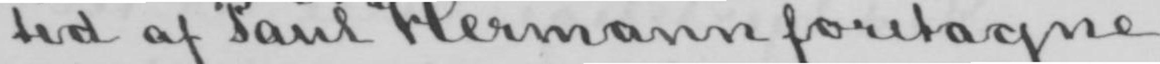tid af Paul Hermann foretagne
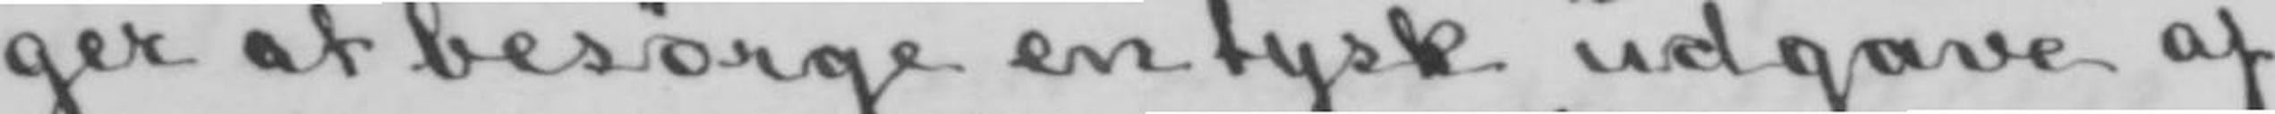ger at besørge en tysk udgave af
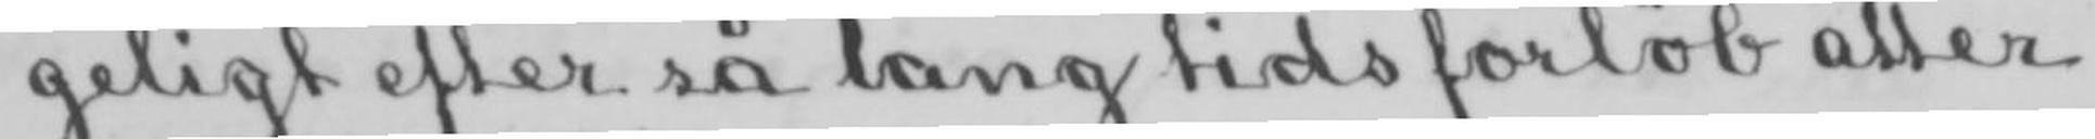geligt efter så lang tids forløb atter
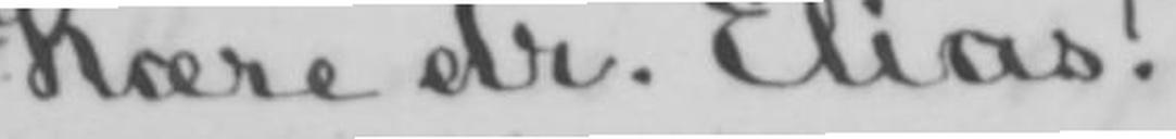Kære dr. Elias!
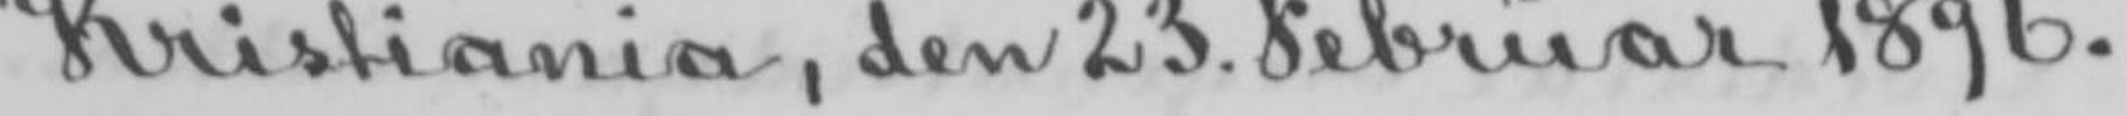Kristiania, den 23. Februar 1896.
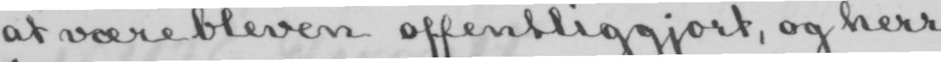at være bleven offentliggjort, og herr
